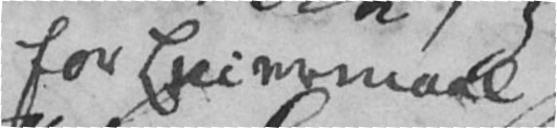for Leiermaal
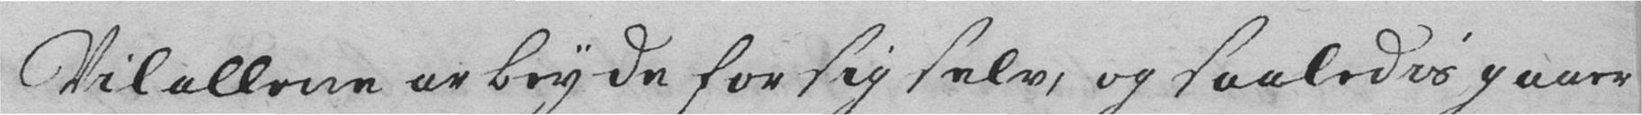Vil allene arbeyde for sig selv, og saaledis gaaer
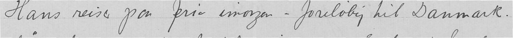Hans reiser paa fri imorgen -foreløbig til Danmark.
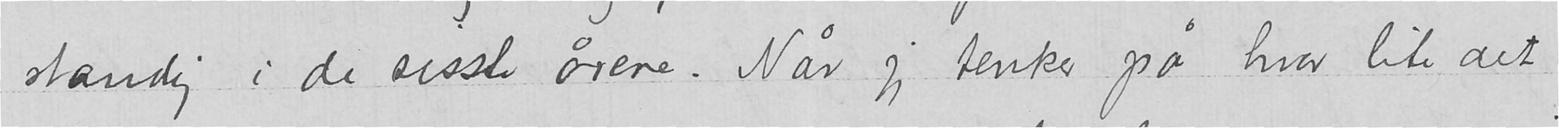standig i de sisste årene. Når jeg tenker pa hvor lite det
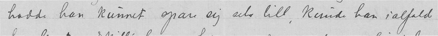hadde han kunnet spare sig selv litt, kunde han ialfald
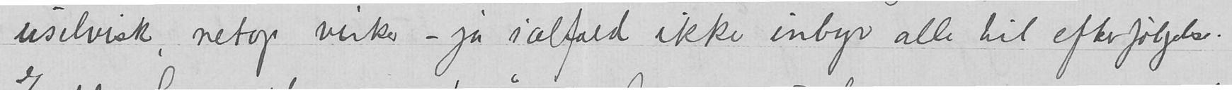uselvisk, netop virker – ja ialfald ikke inbyr alle til efterfølgelse.
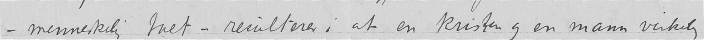menneskelig talt – resulterer i at en kristen og en mann virkelig
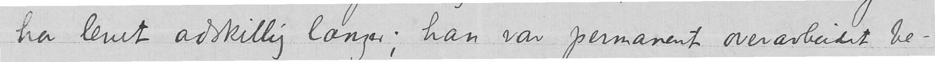har levet adskillig længer han var permanent overarbeidet be-
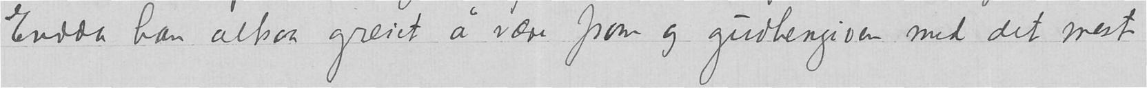Endda han altsaa greiet å være from og gudhengiven med det mest
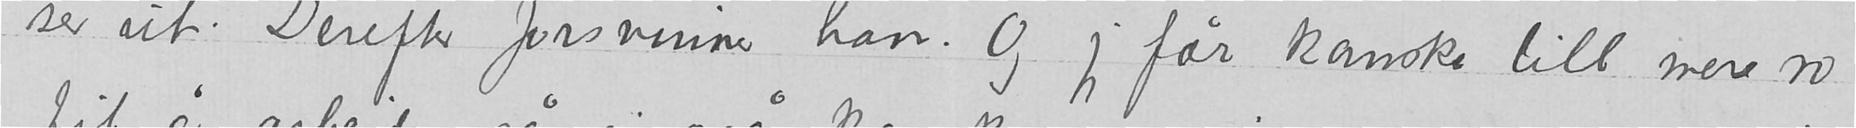ser ut. Derefter forsvinner han. Og jeg får kanske litt mere ro
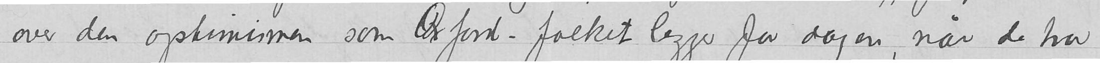over den optimismen som  Aford-folket legger for dagen, nar de tror
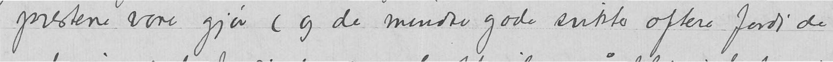prestene vore gjør (og de mindte gode svikter oftere fordi de
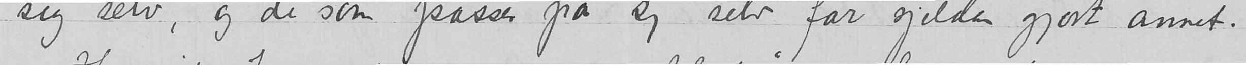sig selv, og de som passer pa sig selv får sjelden gjort annet.
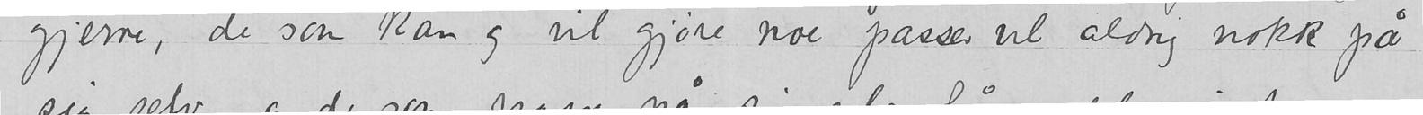gjerne, de som kan og vil gjøre noe passer vel aldrig nokk på
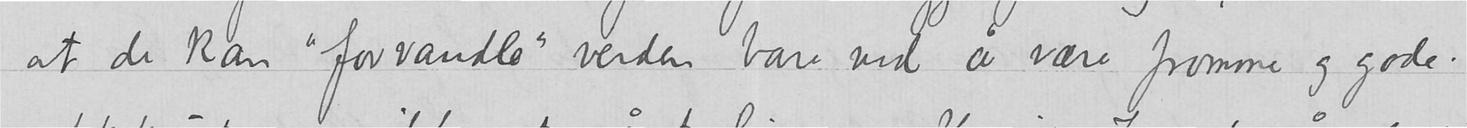at de kan «forvandle» verden bare ved å være fromme og gode.
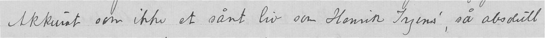Akkurat som ikke et sant liv som Henrik Irgens, så absolutt
